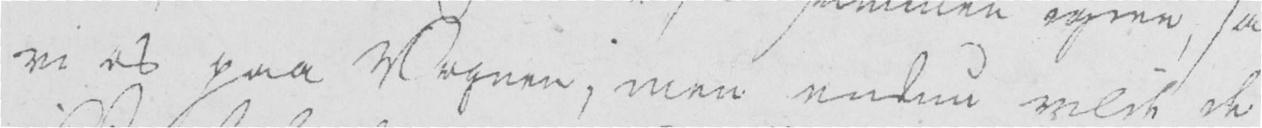vi os paa Vognen, men endnu vilde de
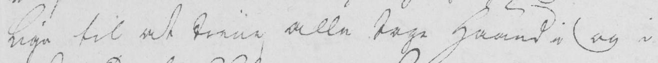lige til at tiene alle tage Haand i og i
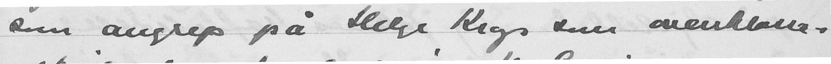som angrep på Helge Krogs som overklasse-
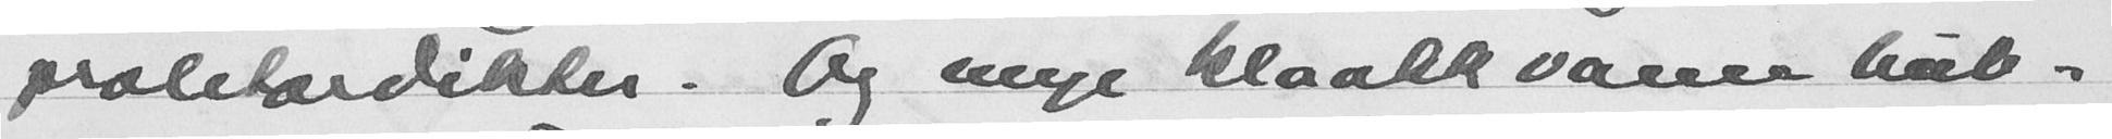prolitærdikter. Og mye kloakkvann bob-
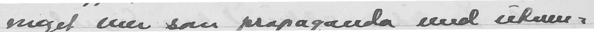meget mer som propaganda med ukue-
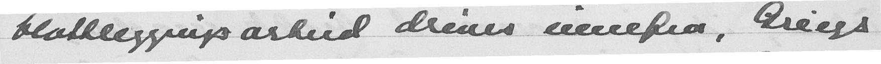blottleggingsarbeid drives innefra, Griegs
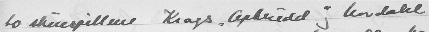to skuespillene Krogs "Opbrudd" og Nordahl
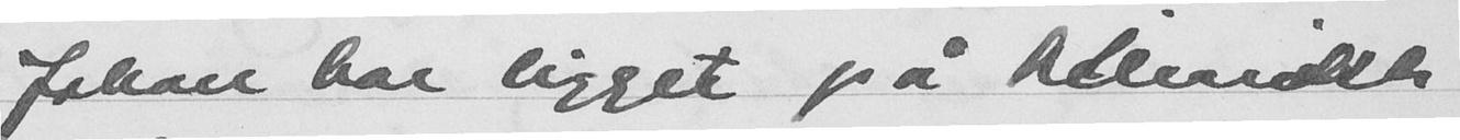Johan har ligget på kllimet
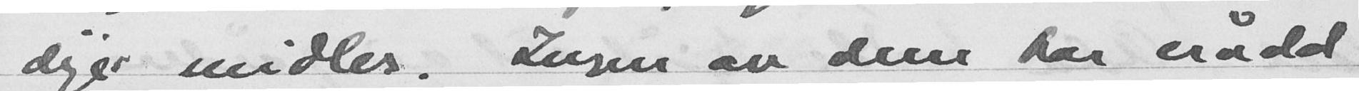lige midler. Ingen av dem har nådd
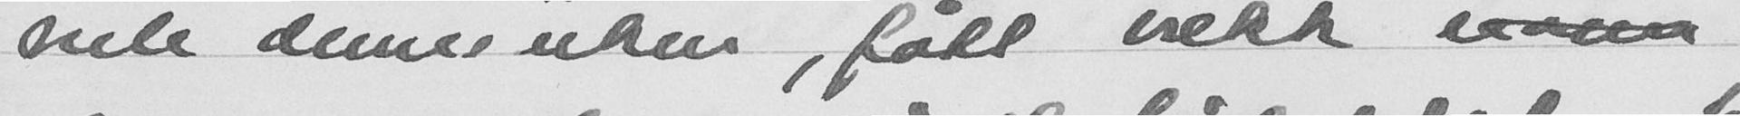hele denee uken, fått vekk
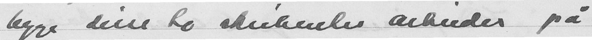begge disse to skribentes arbeider på
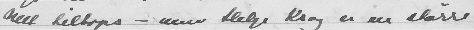helt tiltops - men Helge Krog er en større
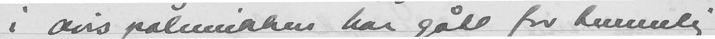i avis polemikkers har gått for temmlig
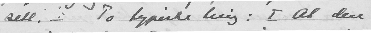sett. - To typiske ting: I At den
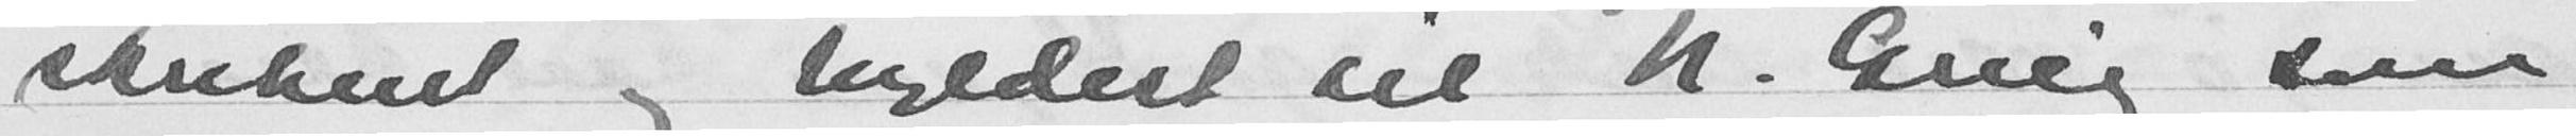skribent og hyldest til N. Grieg som
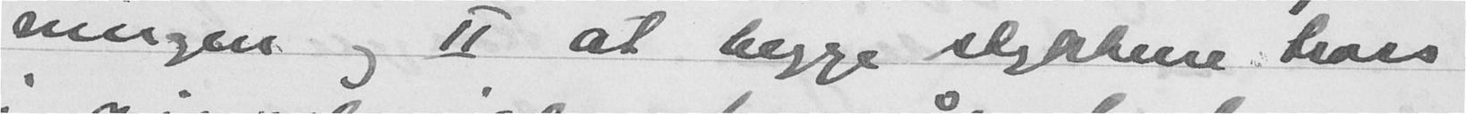nngen og II at begge stykkene tross
